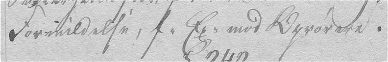Formildelse, f: Ex: mod Oprørere.
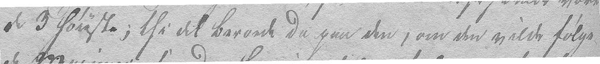de 3 høieste; thi det beroede da paa den, om den vilde følge
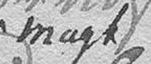Magt
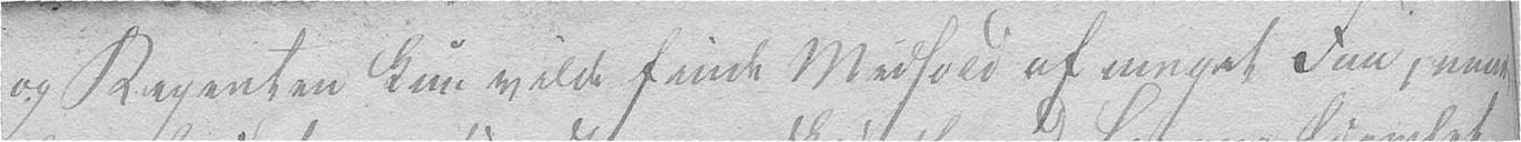og Regenten kun vilde finde Medhold af meget Faa, naar
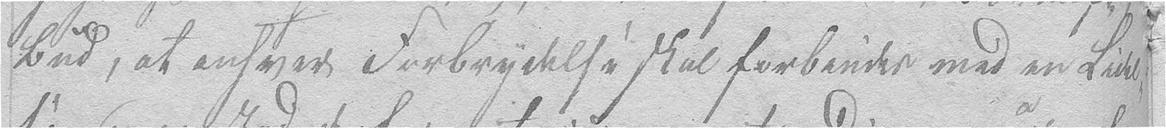bud, at enhver Forbrydelse skal forbindes med en Lidel-
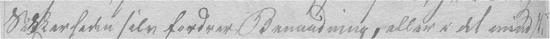Sikkerheden selv fordrer Benaadning, eller i det mindste
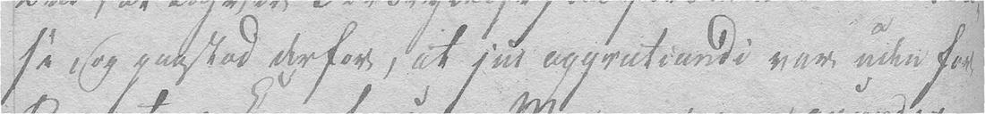se og paastod derfor, at jus aggratiandi var udenfor
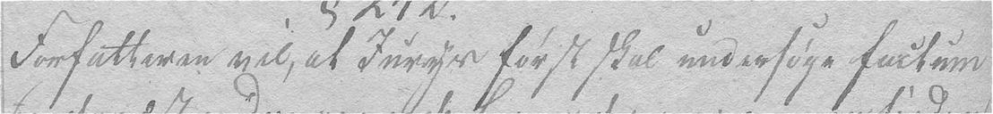Forfatteren vil, at Juryer først skal undersøge factum
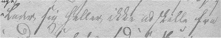lader sig heller ikke adskille fra
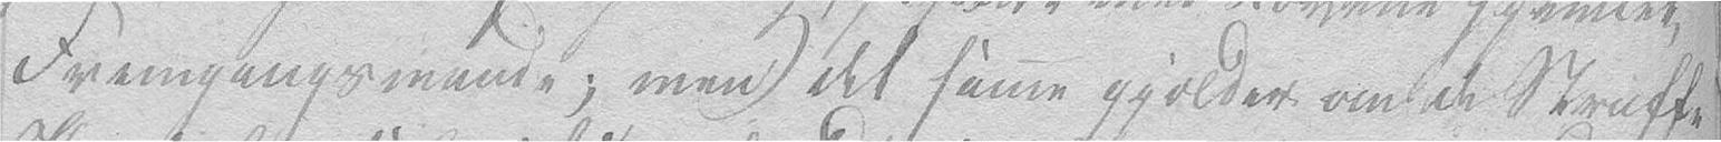Fremgangsmaade; men det samme gjælder om de Straffe
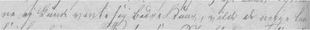ne ey kunde vente sig bedre Kaar, vilde de neppe lade
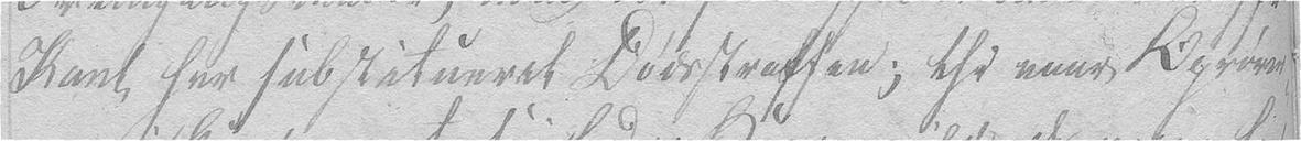Kant har substitueret Dødsstraffen; thi naar Oprører-
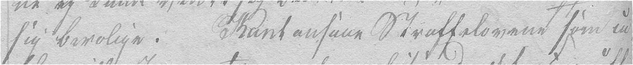sig berolige. Kant ansaae Straffelovene som ca-
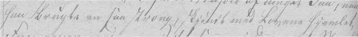han brugte en saa stræng, skjøndt med Lovene hjemlet
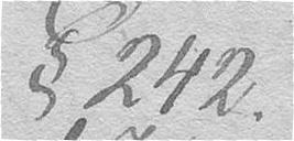§ 242.
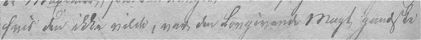hvis den ikke vilde, var den Lovgivende Magt gandske
